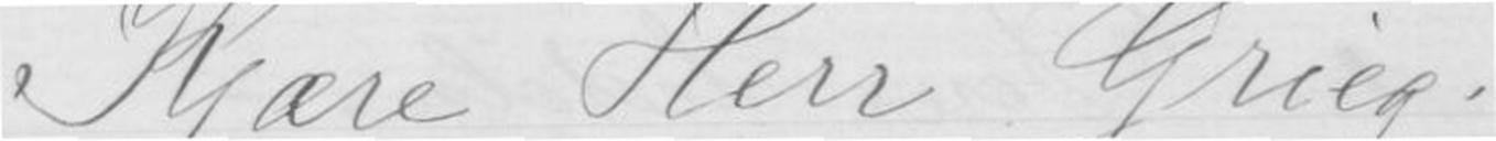Kjære Herr Grieg!
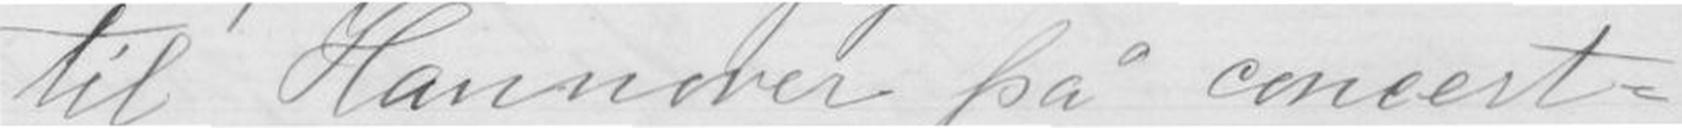til Hannover på concert-
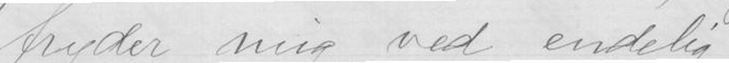fryder mig ved endelig
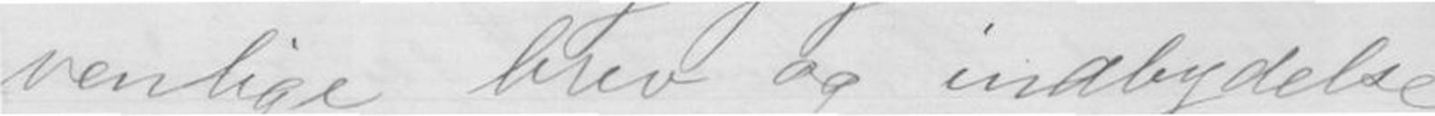venlige brev og indbydelse
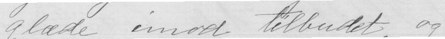glæde imod tilbudet, og
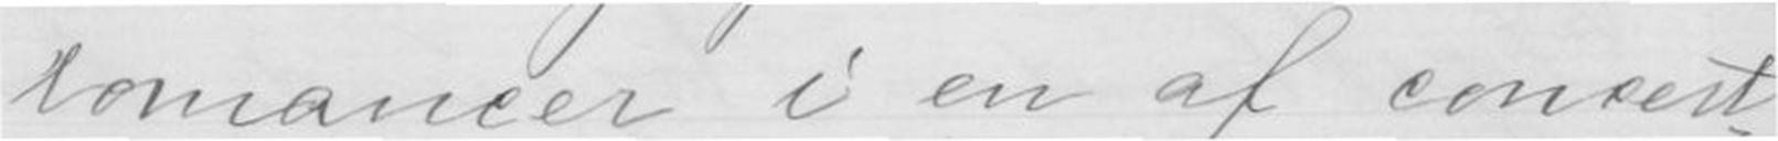romancer i en af concert-
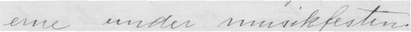erne under musikfesten.
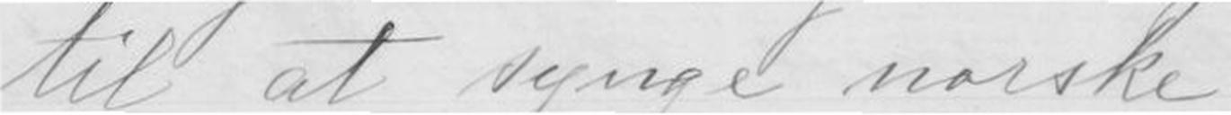til at synge norske
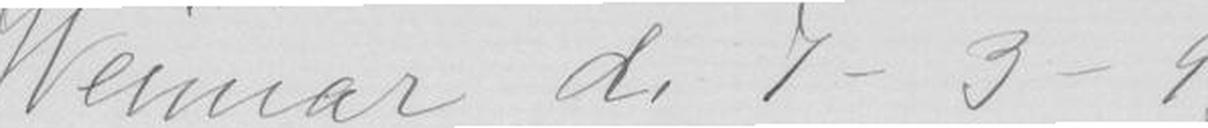Weimar d. 7-3-98
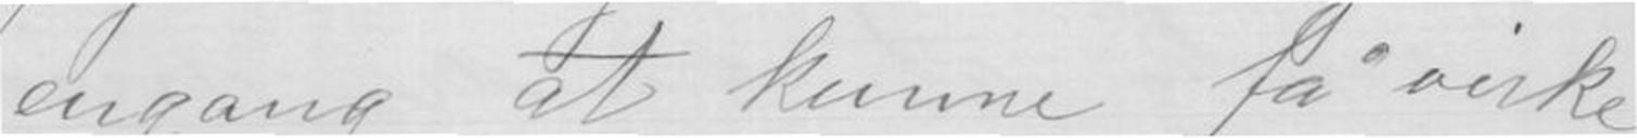engang at kunne få virke
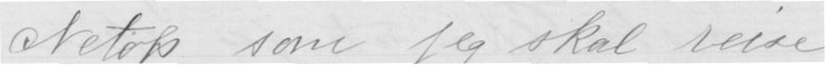Netop som jeg skal reise
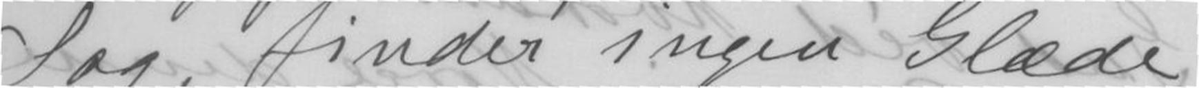Sag, finder ingen Glæde
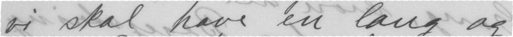vi skal have en lang og
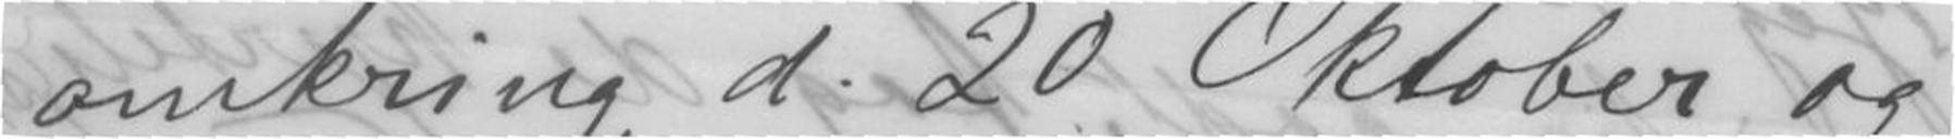omkring d 20 Oktober og
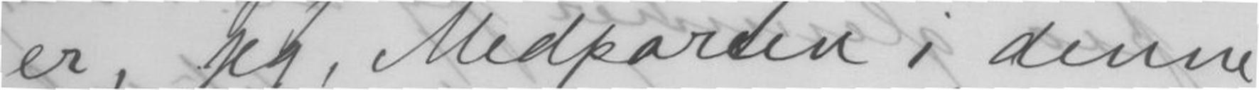er, jeg, Medparten i denne
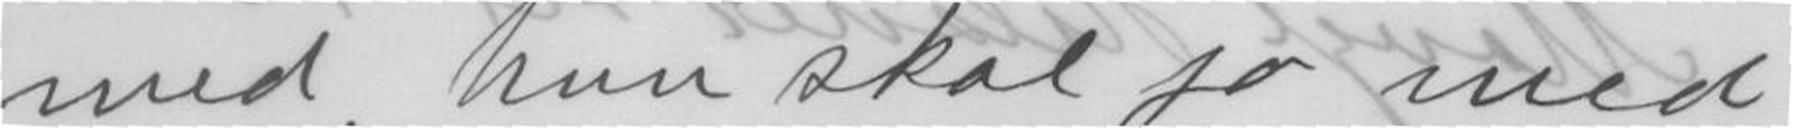med, hun skal jo med
